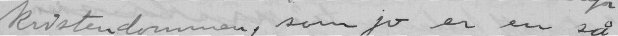kristendommen, som jo er en så
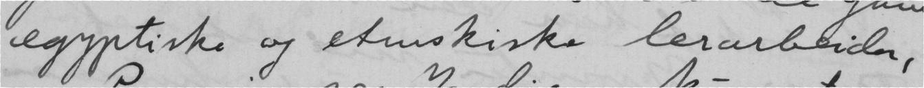egyptiske og etruskiske lerarbeider,
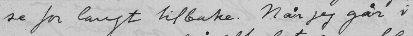se for langt tilbake. Når jeg går i
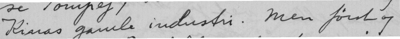Kinas gamle industri. Men først og
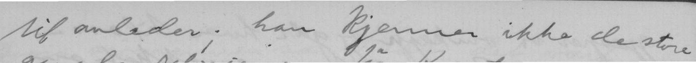til avleder; han kjenner ikke de store
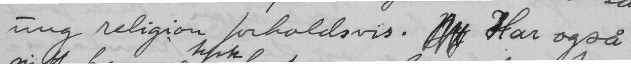ung religion forholdsvis.  Har også
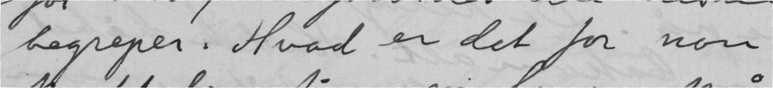begreper. Hvad er det for noen
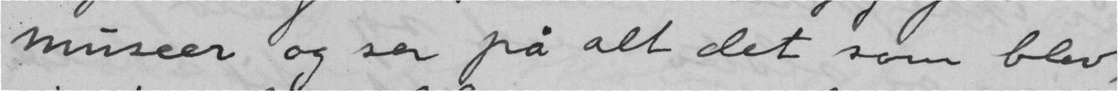museer og ser på alt det som blev,
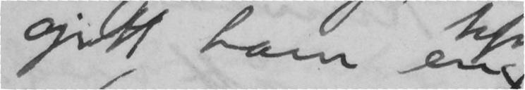gitt ham en
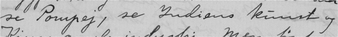se Pompej, se Indiens kunst og
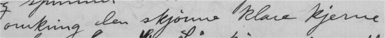omkring den skjønne klare kjerne
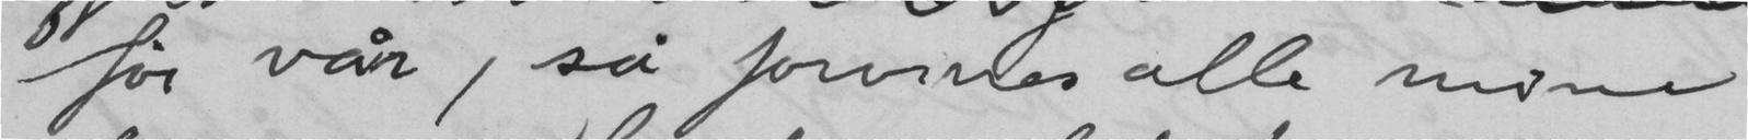før vår, så forsvinder alle mine
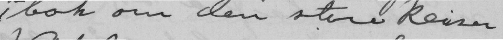bok om den store keiser
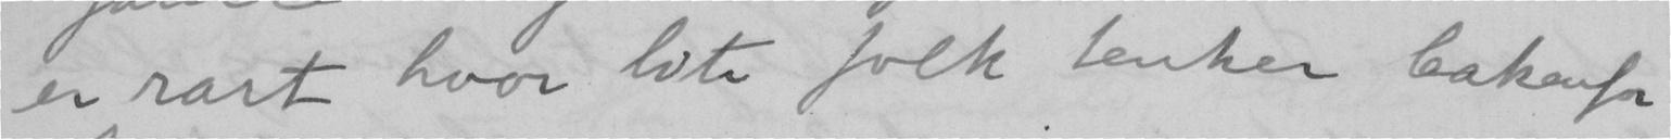er rart hvor lite folk tenker bakenfor
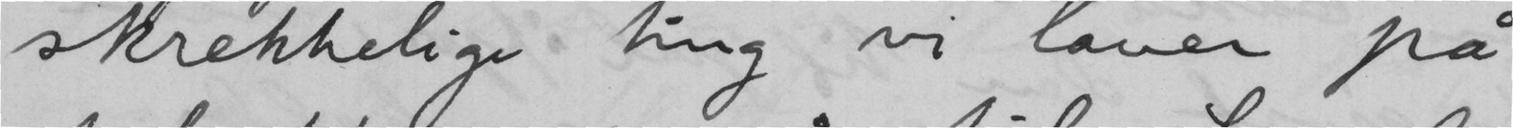skrekkelige ting vi laver på
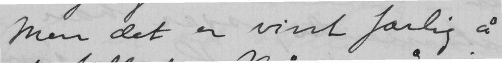Men det er visst farlig å
